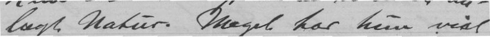lagt Natur. Meget har hun vist
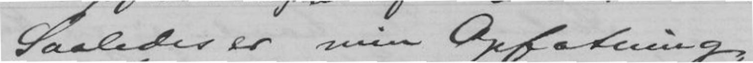Saaledes er min Opfatning
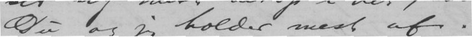Du og jeg holder mest af.
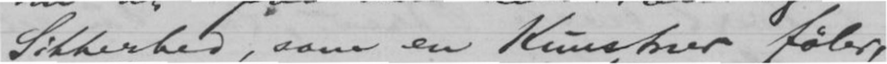Sikkerhed, som en Kunstner føler,
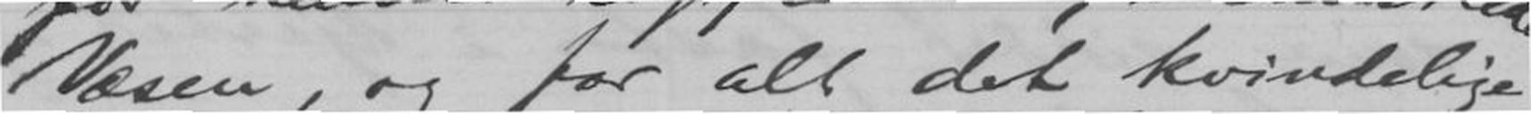Væsen, og for alt det kvindelige
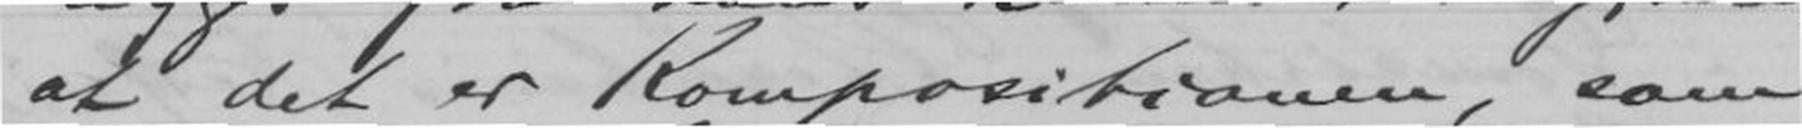at det er Kompositionen, som
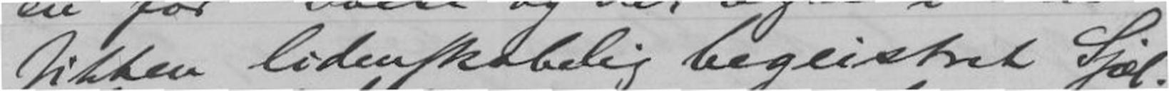sikken lidenskabelig begeistret Sjæl.
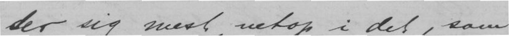der sig mest netop i det, som
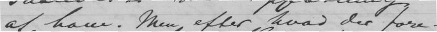af ham. Men efter hvad der fore-
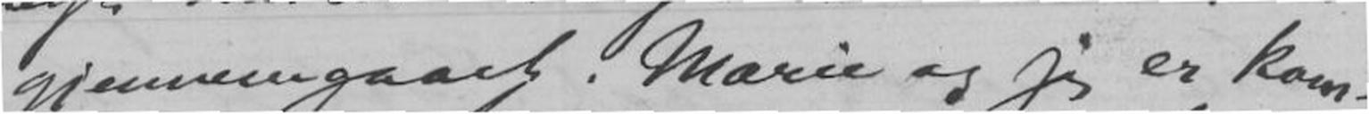gjennemgaaet. Marie og jeg er kom-
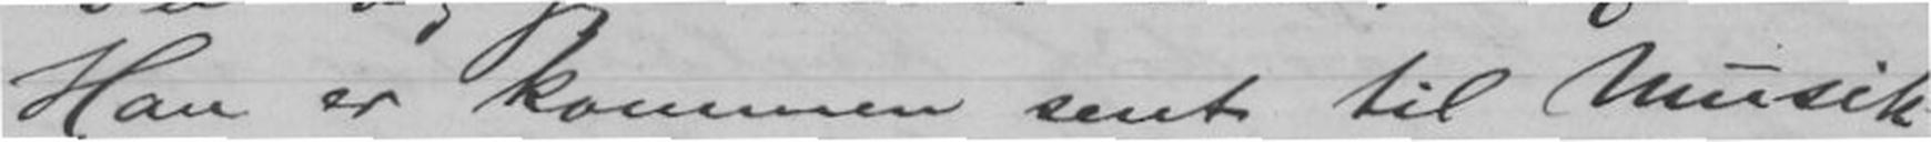Han er kommen sent til Musik-
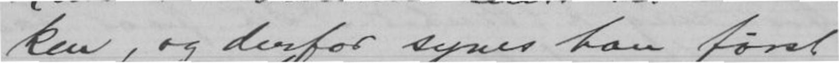ken, og derfor synes han først
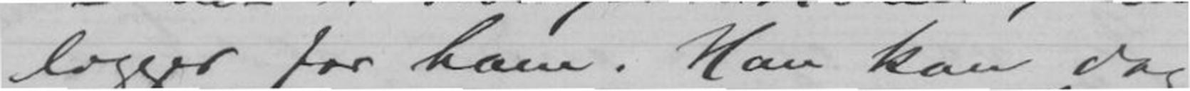ligger for ham. Han kan dog
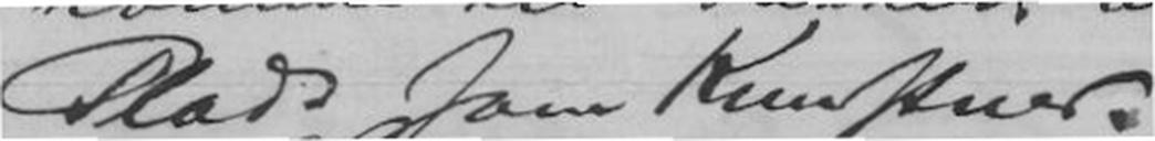Plads som Kunstner.
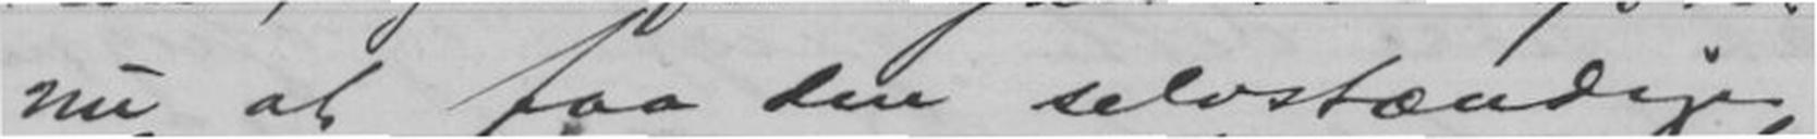nu at faa den selvstændige
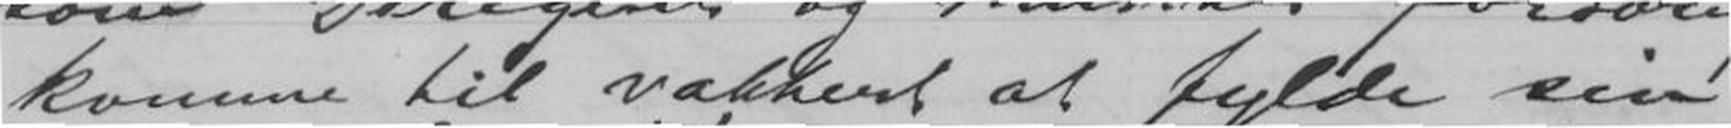komme til vakkert at fylde sin
